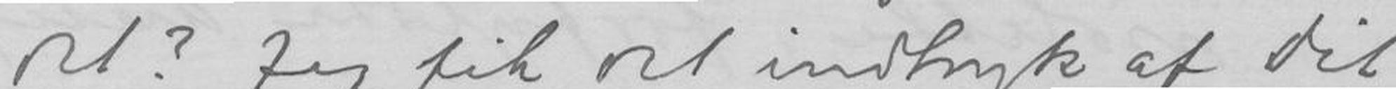det? Jeg fik det indtryk af dit
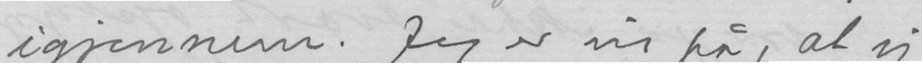igjennem. Jeg er vis på, at vi
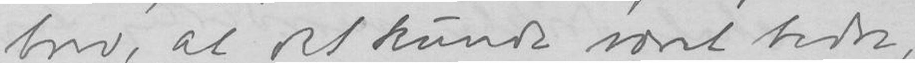brev, at det kunde været bedre,
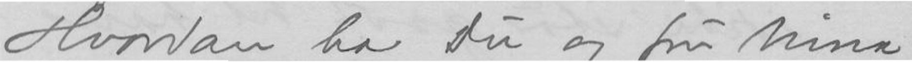Hvordan har du og Fru Nina
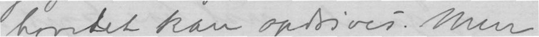hovedet kan opdrives. Men
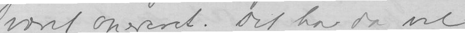været opereret. Det har da vel
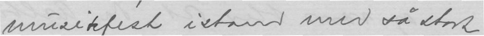musikfest istand med så stor
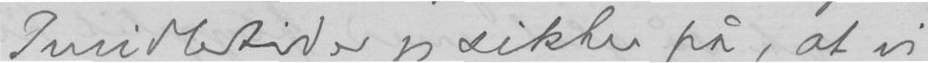Imidlertid er jeg sikker på, at vi
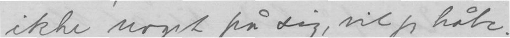ikke noget på sig, vil jeg håbe.
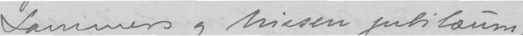Lammers og Nissen jubilæum
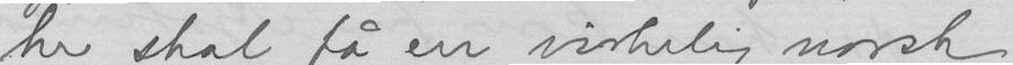her skal få en virkelig norsk
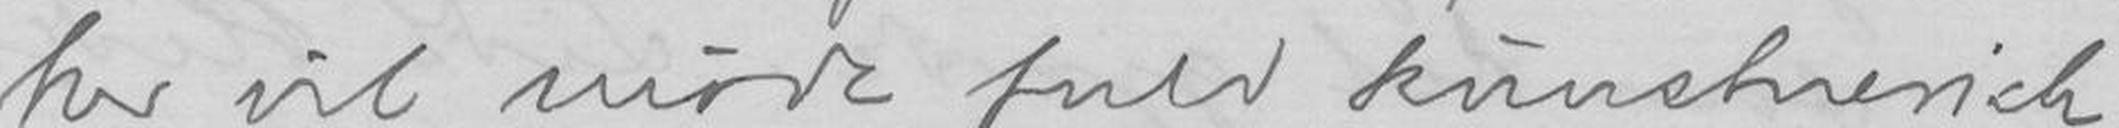her vil møde fuld kunstnerisk
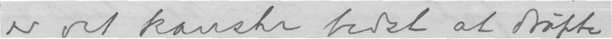er det kanske bedst at drøfte
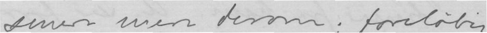senere mere derom; foreløbig
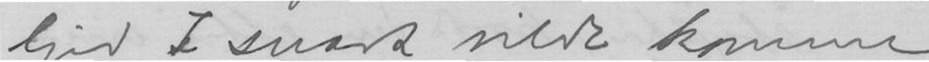Giv I snart vilde komme
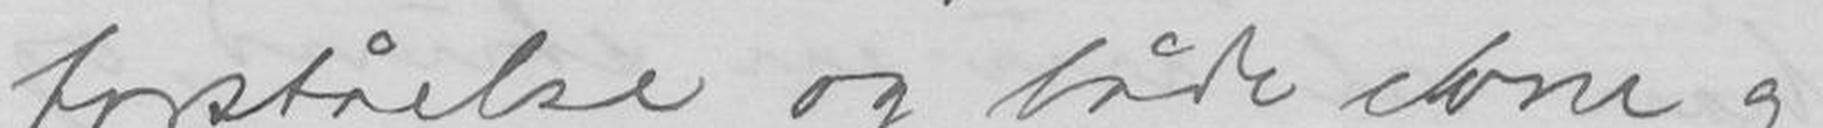forståelse og både evne og
